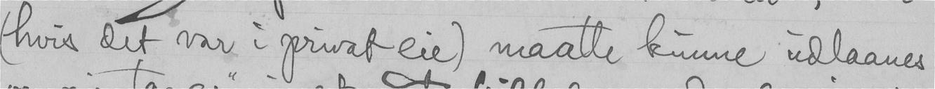(hvis det var i privat eie) maatte kunne udlaanes
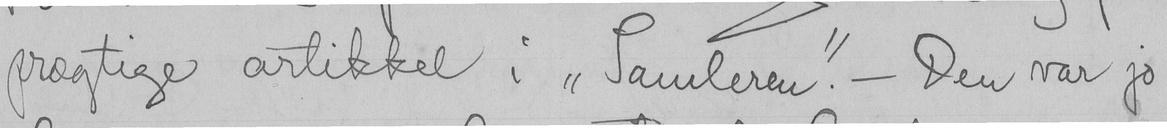prægtige artikkel i "Samleren". - Den var jo
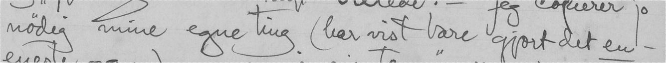nødig mine egne ting (har vist bare gjort det en -
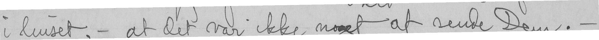i huset, - at det var ikke nogt at sende Dem. -
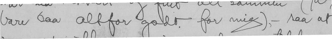bare saa altfor godt for mig), - saa at
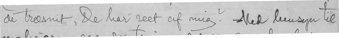de træsnit, De har seet af mig. Med hensyn til
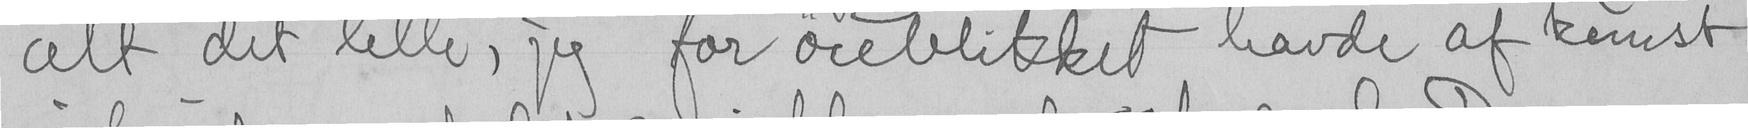alt det lille, jeg for øieblikket havde af kunst
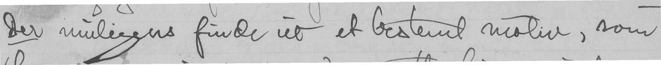der muligens finde ut et bestemt motiv, som
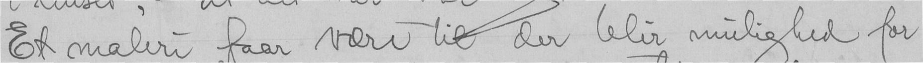Et maleri faar være til der blir mulighed for
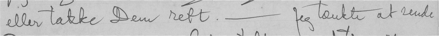eller takke Dem reft. - Jeg tænkte at sende
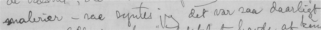malerier - saa syntes jeg det var saa daarligt
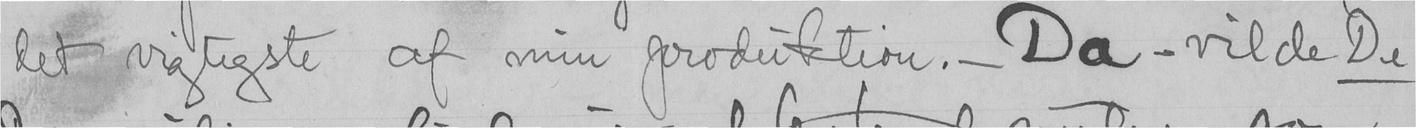det vigtigste af min produktion. - Da - vilde De
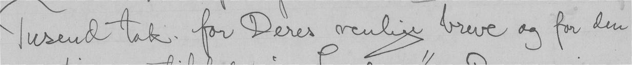Tusend tak for Deres venlige breve og for den
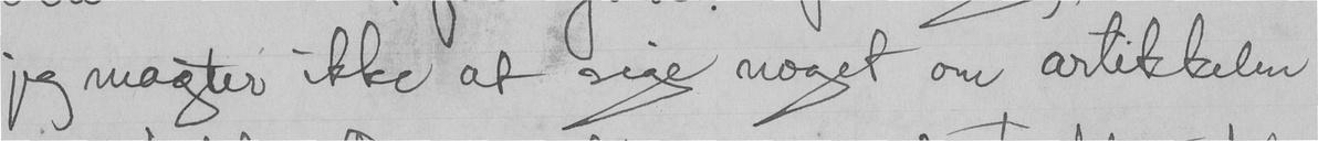jeg magter ikke at sige noget om artikkelen
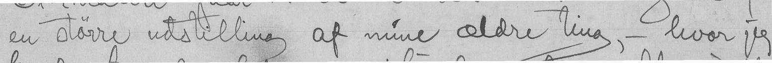en større udstilling af mine ældre ting, - hvor jeg
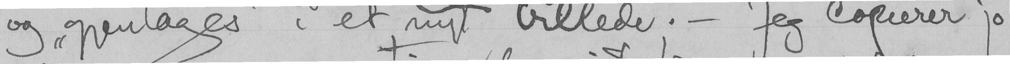og "gjentages" i et nyt billede. - Jeg copierer j
